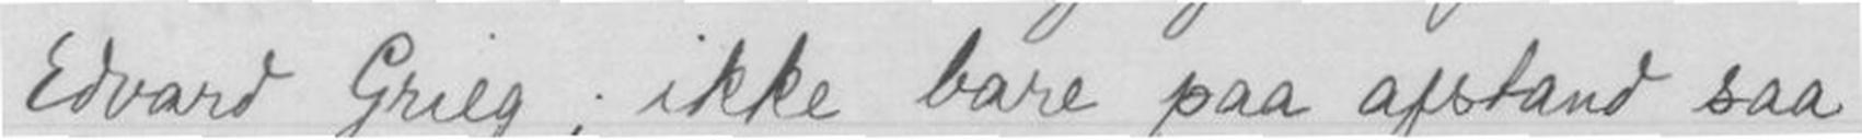Edvard Grieg; ikke bare paa afstand saa
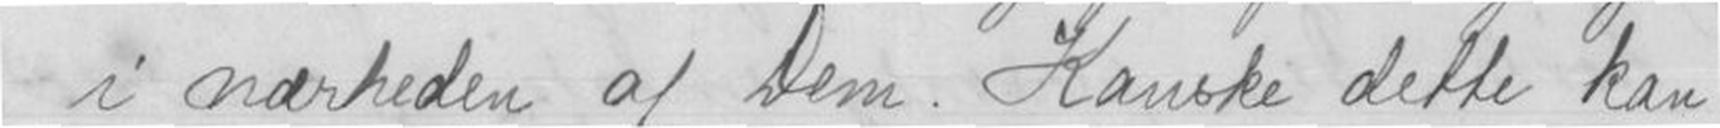i nærheden af Dem. Kanske dette kan
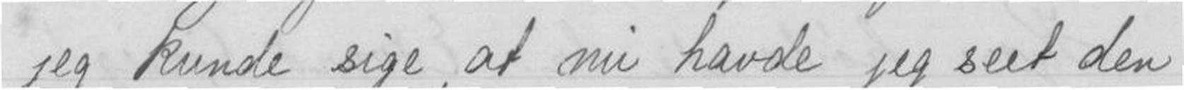jeg kunde sige, at nu havde jeg seet den
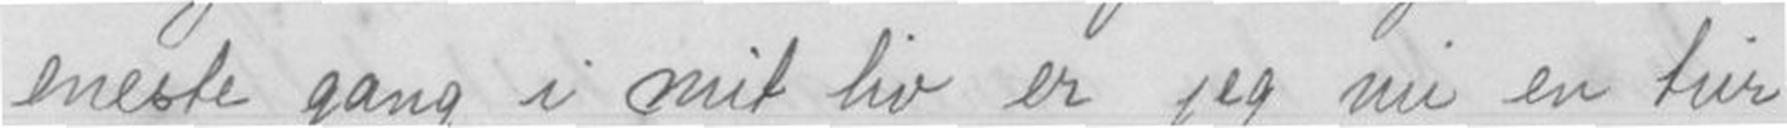eneste gang i mit liv er jeg nu en tur
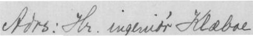Adrs: Hr. ingeniør Klæboe
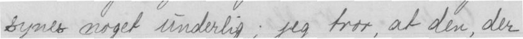synes noget underlig; jeg tror, at den, der
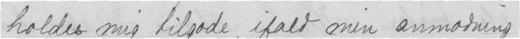holdes mig tilgode, ifald min anmodning
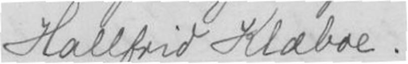Hallfrid Klæboe.
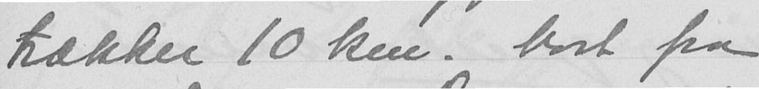trække 10 km. bort fra
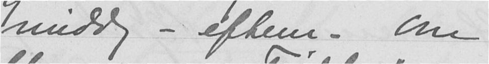middag - efterm. Om
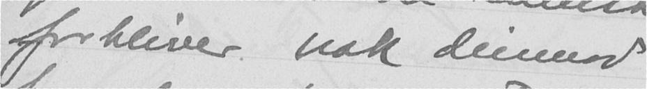forbliver nok derimod
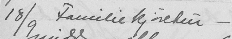18/9 Familiekjøretur -
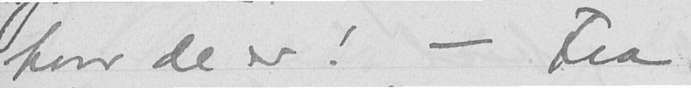hvor de er! - Fra
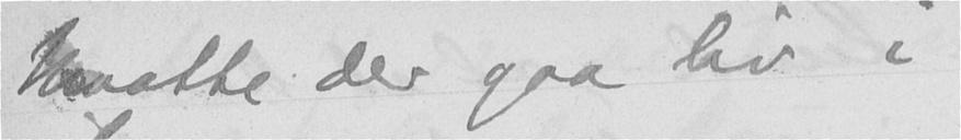Maatte der gaa liv i
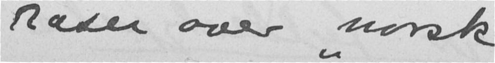raser over "norsk
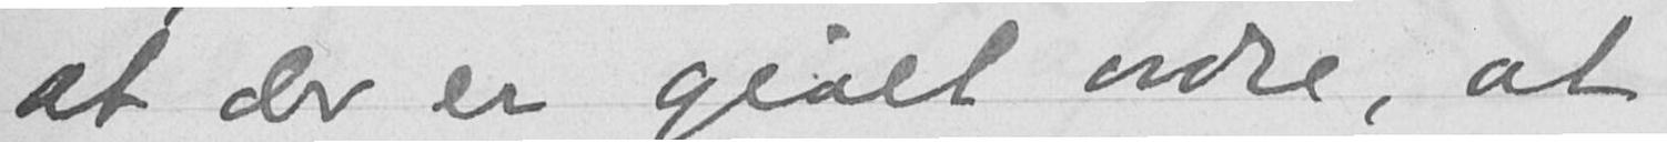at der er givet ordre, at
